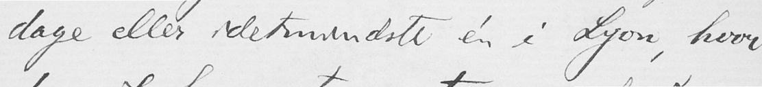dage eller idetmindste én i Lyon, hvor
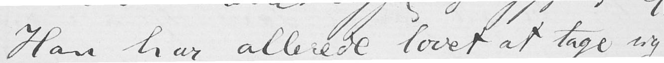Han har allerede lovet at tage sig
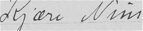Kjære Nini,
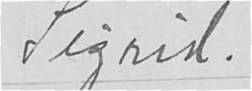Sigrid
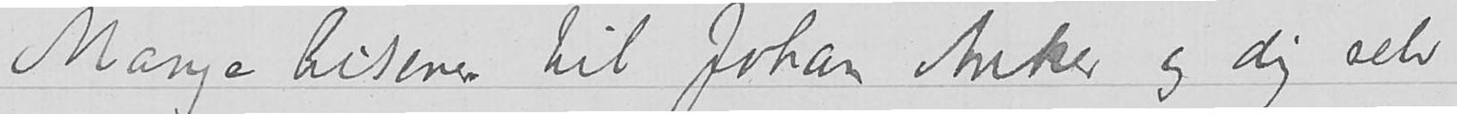Mange hilsener til Johan Anker og dig selv
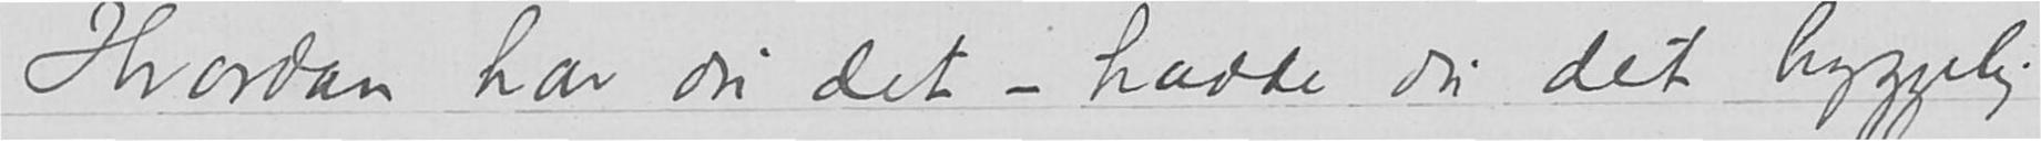Hvordan har du det – hadde du det hyggelig
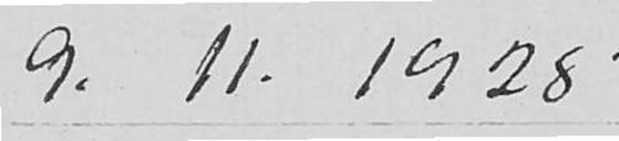9.11.1928
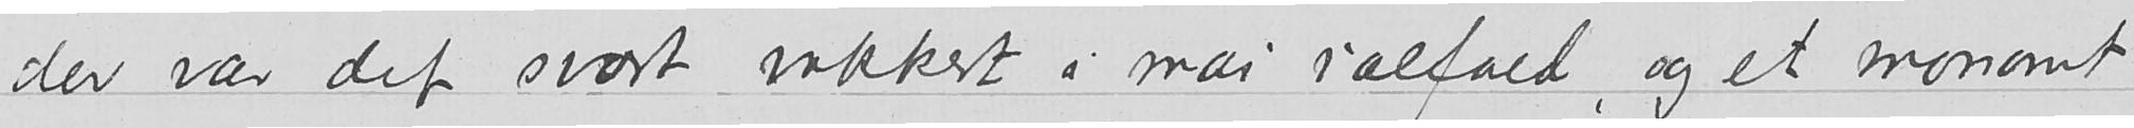der var det svært vakkert i mai ialfald, og et morsomt
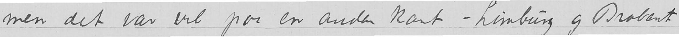men det var vel paa en anden kant – Limburg og Brabant
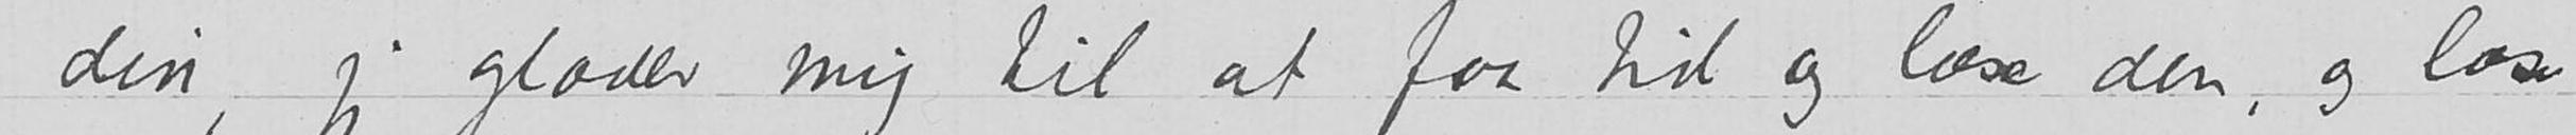jeg glæder mig til at faa tid og læse den, og læse
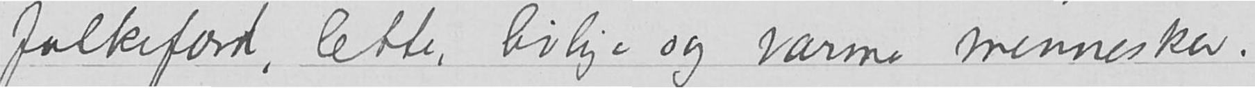folkefærd, lette, livlige og varme mennesker.
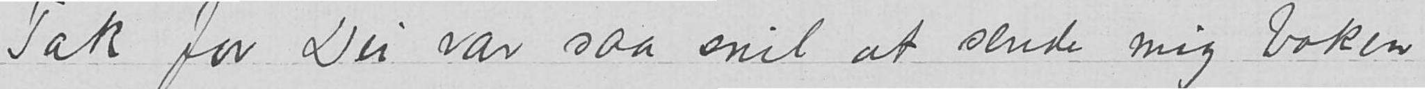Tak for Du var saa snil at sende mig boken din
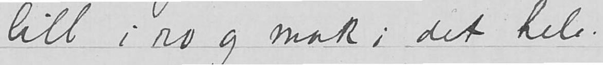litt i ro og mak i det hele.
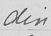din
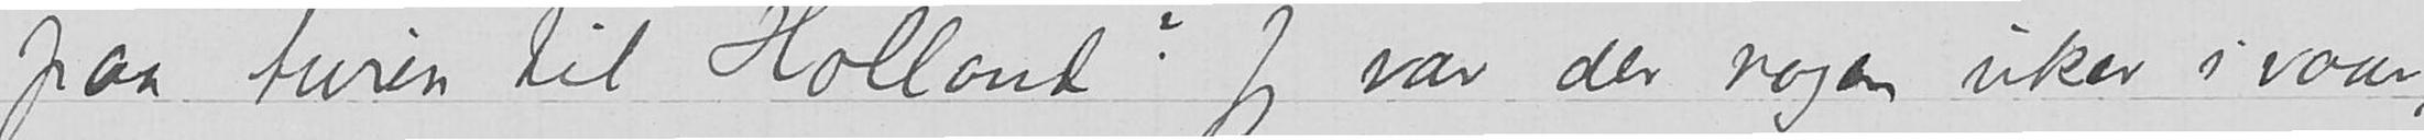paa turen til Holland? Jeg var der nogen uker i vaar,
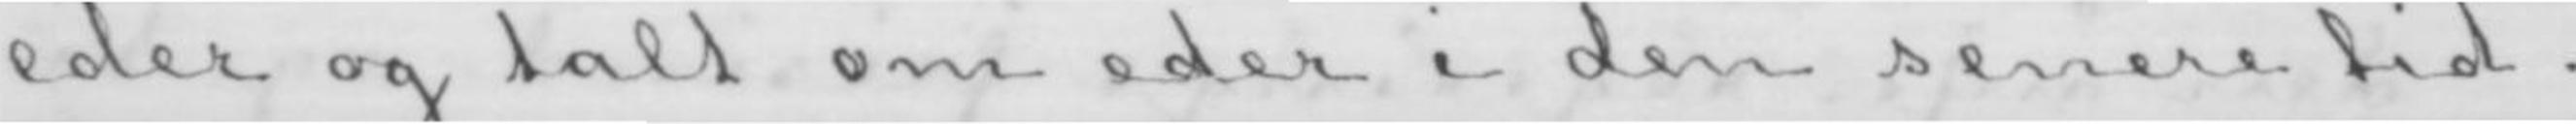eder og talt om eder i den senere tid.
